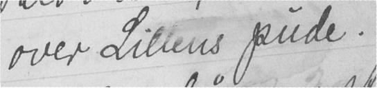over Lillens pude.
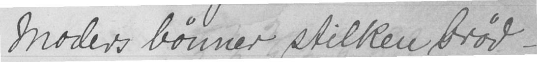Moders bønner stilken brød -
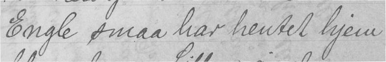Engle smaa har hentet hjem
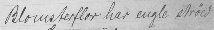Blomsterflor har engle strøed
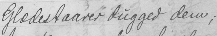Glædestaarer dugged dem;
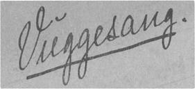Vuggesang.
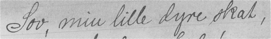Sov, min lille dyre skat,
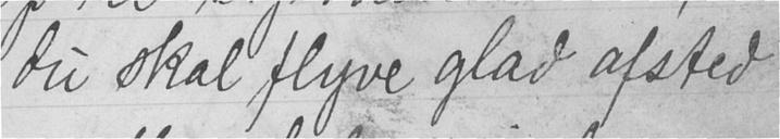du skal flyve glad afsted
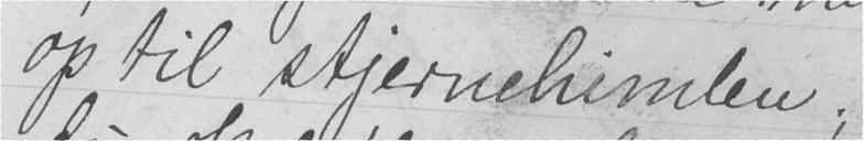op til stjernehimlen;
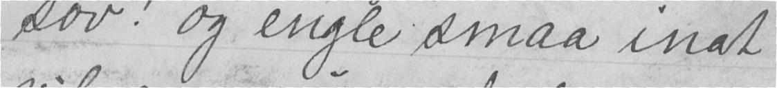sov! og engle smaa inat
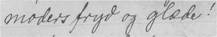moders fryd og glæde!
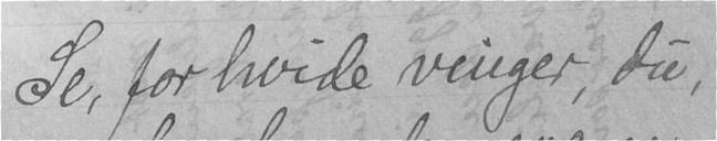Se, for hvide vinger, du,
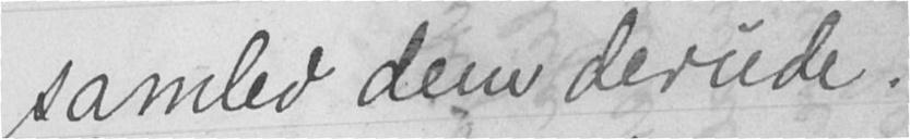samled dem derude.
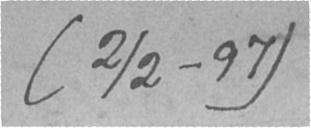(2/2-97)
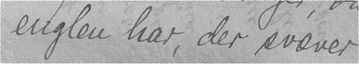englen har, der svæver
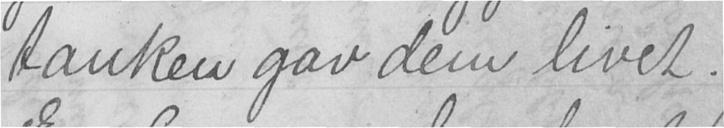tanken gav dem livet.
